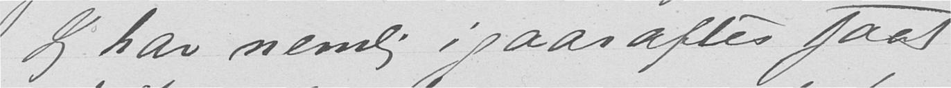Jeg har nemlig igaaraftes faat
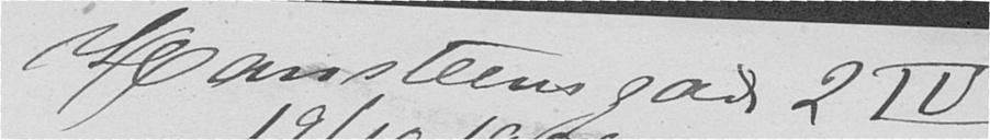Hansteensgade 2 IV
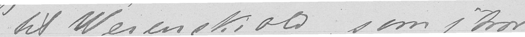til Werenskiold, som jeg tror
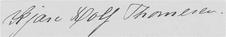Kjære Rolf Thomesen.
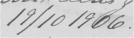19/10 1906.
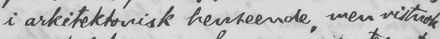i arkitektonisk henseende, men vistnok
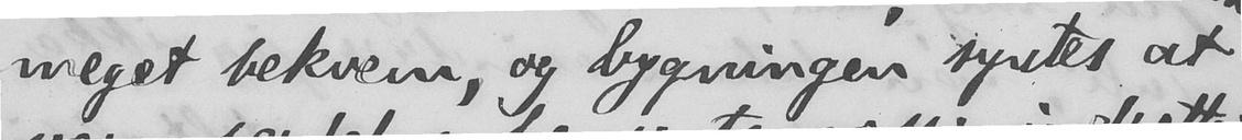meget bekvem, og bygningen syntes at
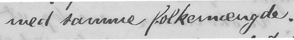med samme folkemængde.
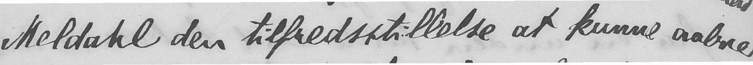Meldahl den tilfredsstillelse at kunne aabne
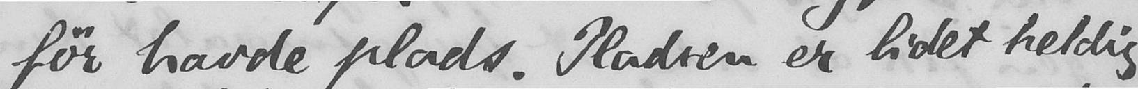før havde plads. Pladsen er lidet heldig
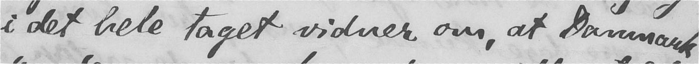i det hele taget vidner om, at Danmark
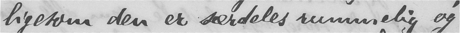ligesom den er særdeles rummelig og
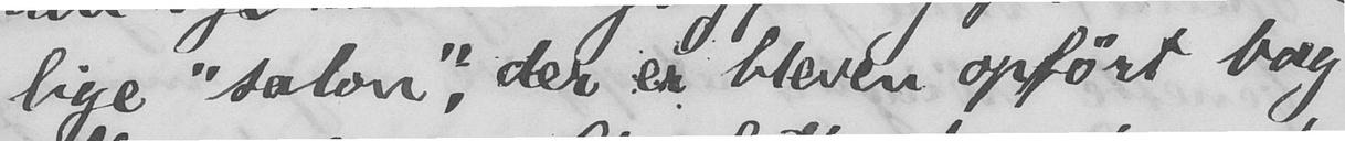lige "salon", der er bleven opført bag
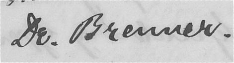Dr. Brenner.
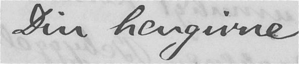Din hengivne
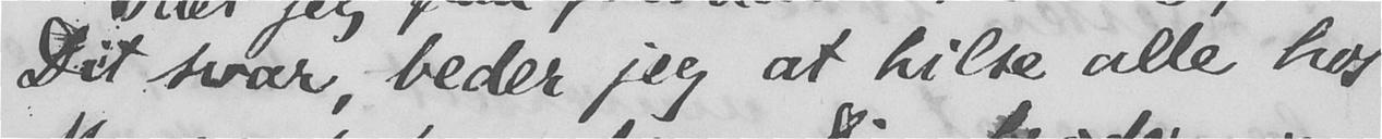Dit svar, beder jeg at hilse alle hos
